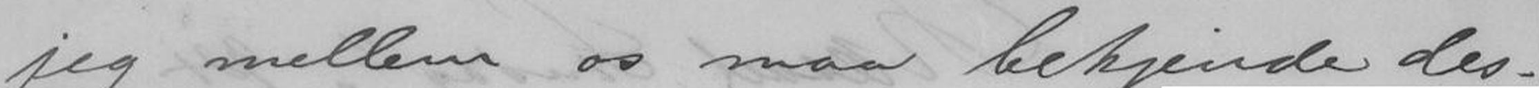jeg mellem os maa bekjende des-
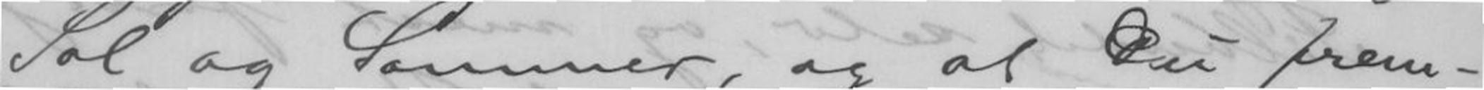Sol og Sommer, og at Du frem-
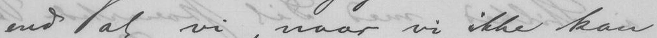end at vi, naar vi ikke kan
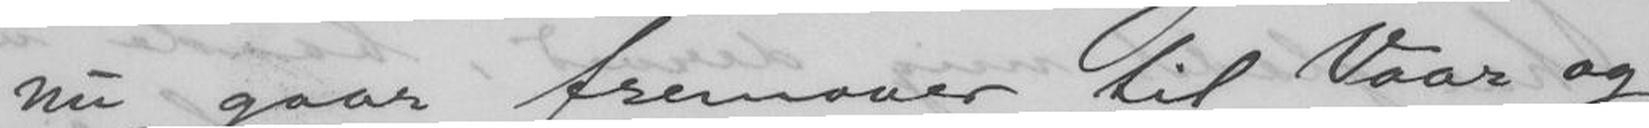nu gaar fremover til Vaar og
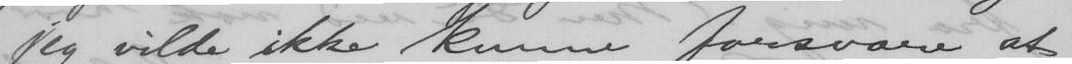jeg vilde ikke kunne forsvare at
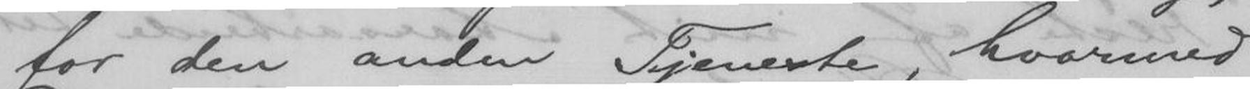for den anden Tjeneste, hvormed
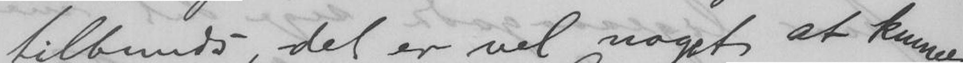tilbunds, det er vel noget at kunne
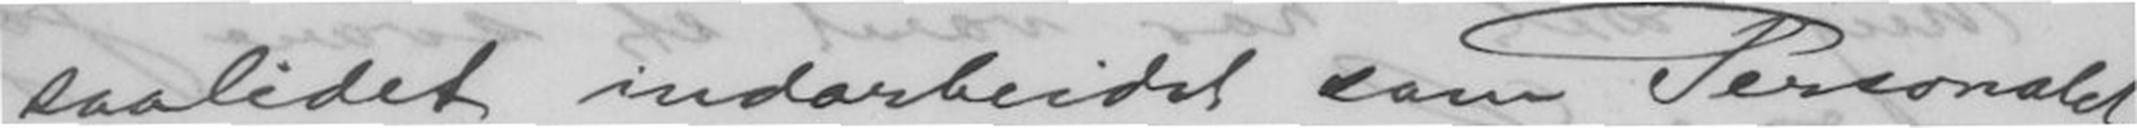saalidet indarbeidet som Personalet
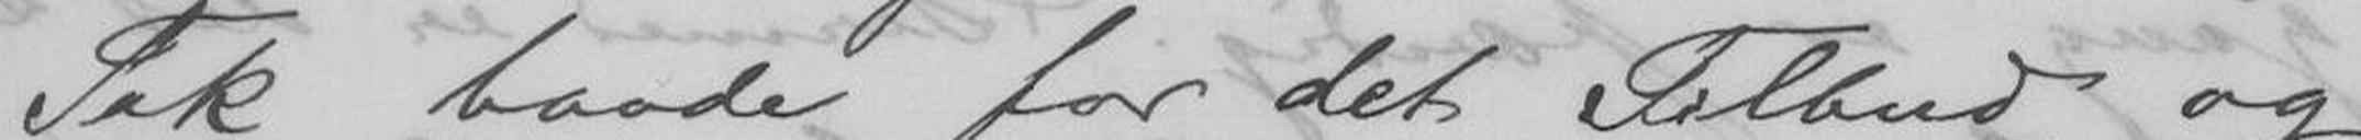Tak baade for det Tilbud og
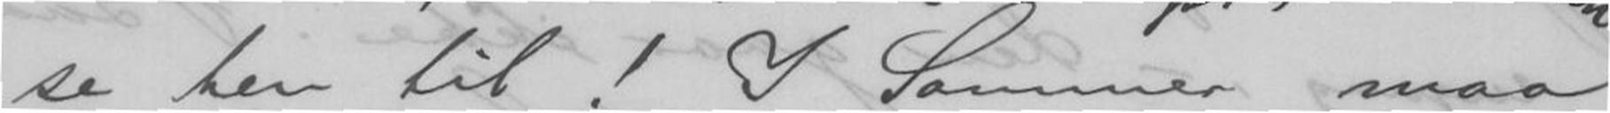se hen til! I Sommer maa
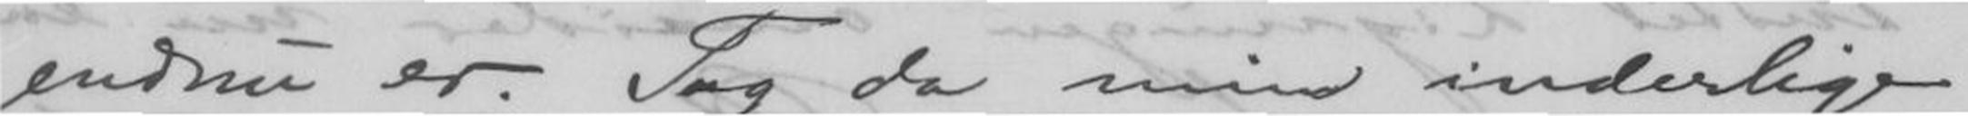endnu er. Tag da min inderlige
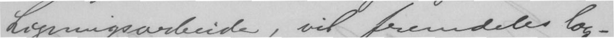Ligningsarbeide, vil fremdeles læg-
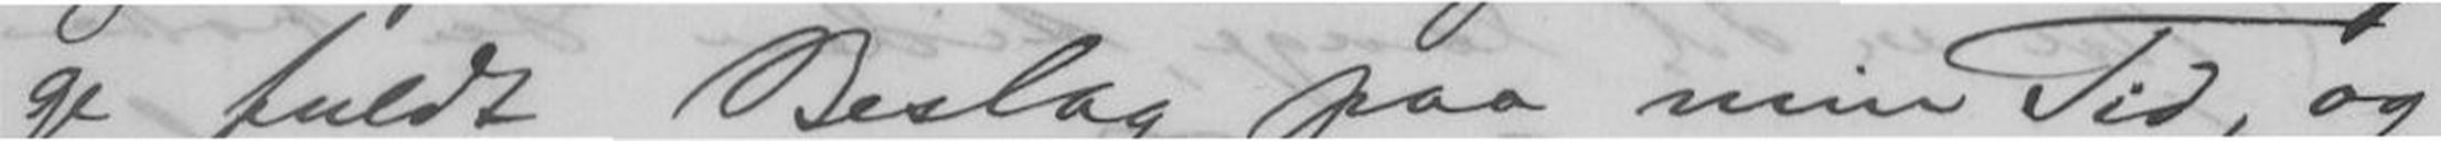ge fuldt Beslag paa min Tid, og
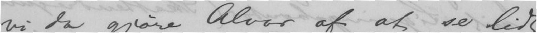vi da gjøre Alvor af at se lidt
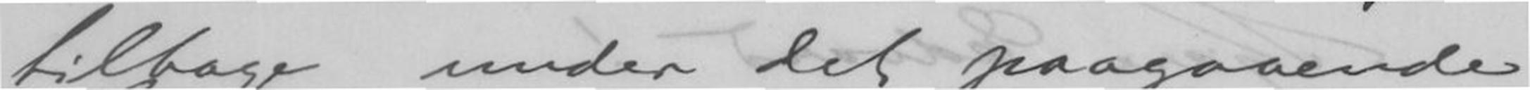tilbage under det paagaaende
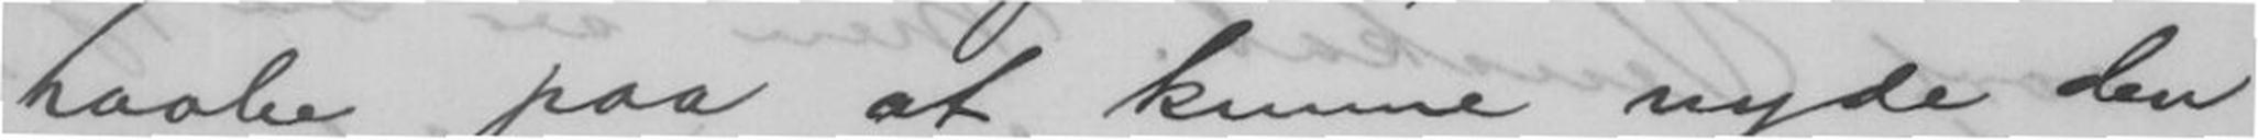haabe paa at kunne nyde den
